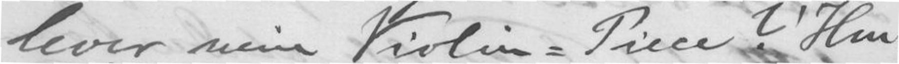lever mine Violin-Piece? Hm!
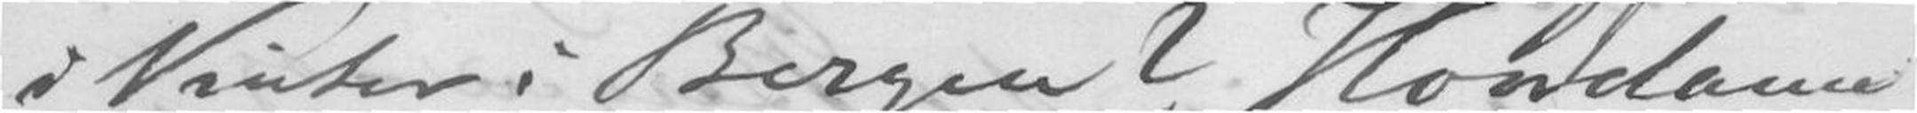i Vinter i Bergen? Hvordann
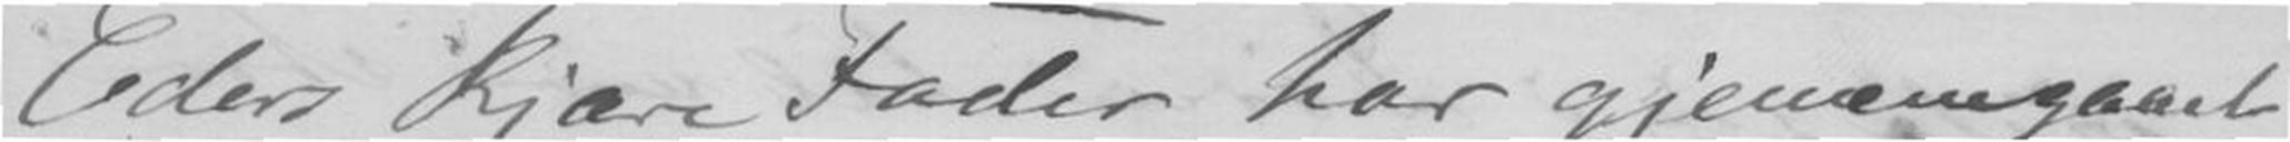Eders kjære Fader har gjennemgaaet
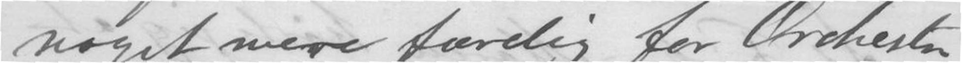noget mere færdig for Orchester
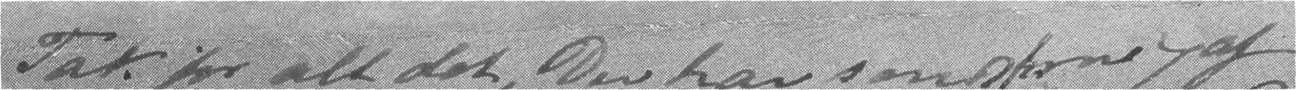Tak for alt det, Du har sandstene af
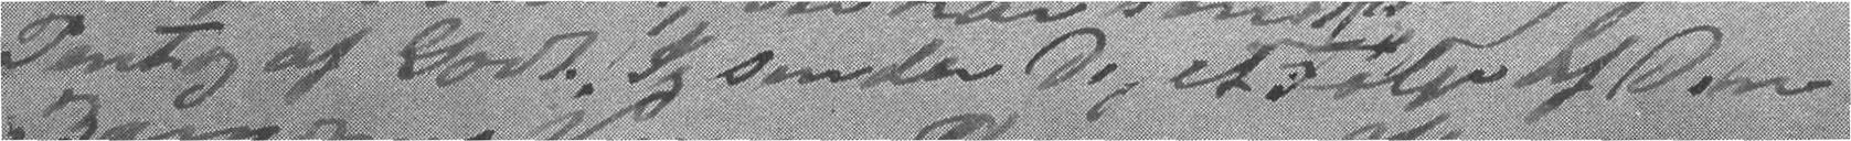Pent og af Godt! Jeg sender dig et Følge af Dine
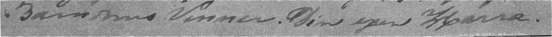Barndoms Venner. Din egen Harra
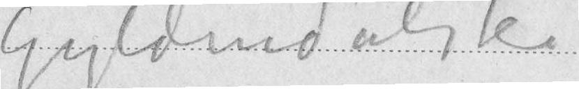Gyldendalske
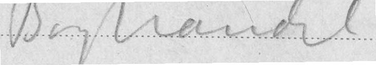Boghandel
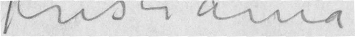Kristiania
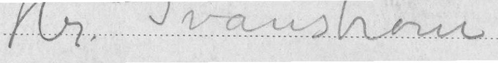Hr. Svanström
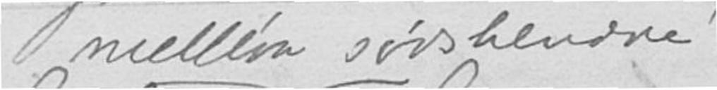mellem søskendne
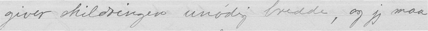giver skildringen unødig bredde, og jeg maa
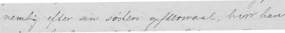nemlig efter sin søsters giftermaal, hvor han
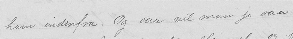ham indenfra. Og saa vil man jo saa
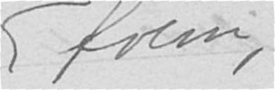frem,
